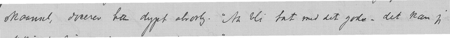skaansel, svarer han dypt alvorlig. «Aat bli tat med det gode – det kan jeg
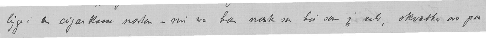ligge i en cigarkasse næsten – nu er han næsten saa høi som jeg selv, skvætter over paa
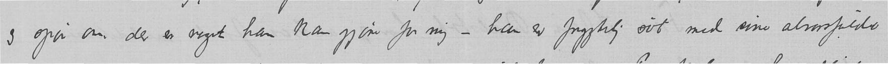og spør om der er noget han kan gjøre for mig – han er frygtelig søt med sine alvorsfulde
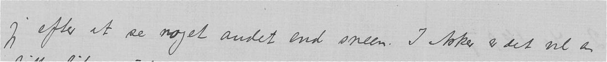jeg efter at se noget andet end sneen. I Asker er det vel en
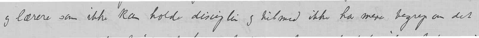og lærere som ikke kan holde disciplin og til med ikke har mere begrep om det
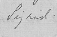Sigrid.
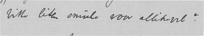bitte liten smule vaar allikevel?
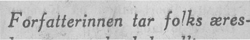Forfatterinnen tar folks æres-
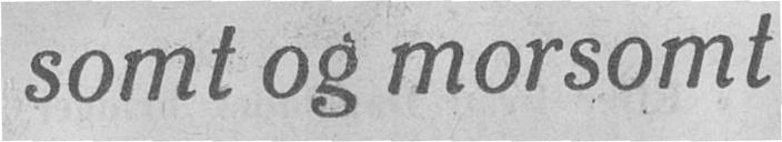somt og morsomt"
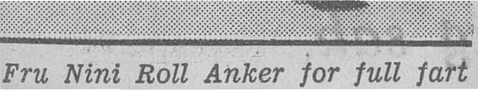Fru Nini Roll Anker for full fart
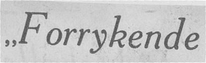"Forrykende
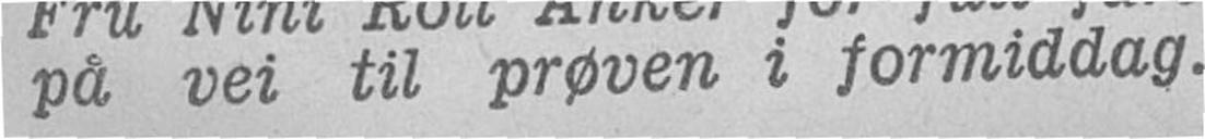på vei til prøven i formiddag.
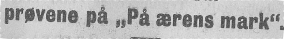prøvene på "På ærens mark".
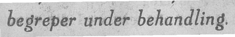begreper under behandling.
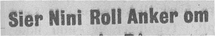Sier Nini Roll Anker om
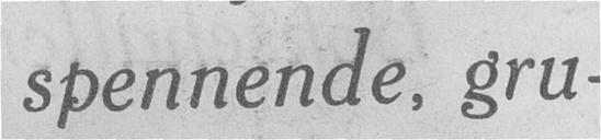spennende, gru-
